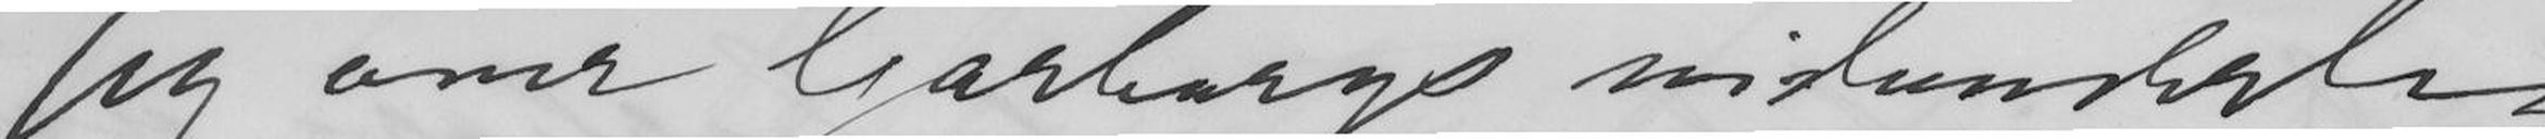jeg over Garborg vidunderlige
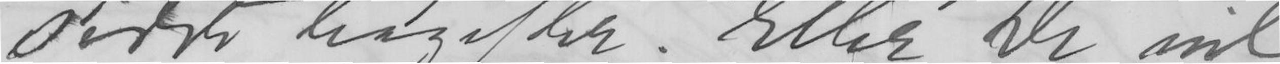sidste bagefter. Eller De vil
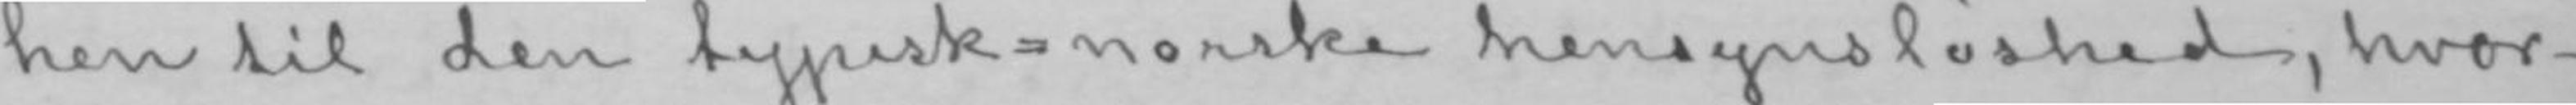hen til den typisk-norske hensynsløshed, hvor-
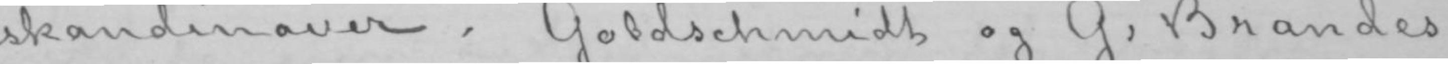skandinaver. Goldschmidt og G: Brandes
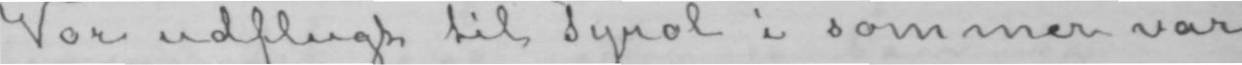Vor udflugt til Tyrol i sommer var
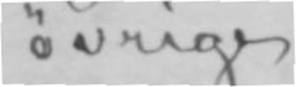øvrige.
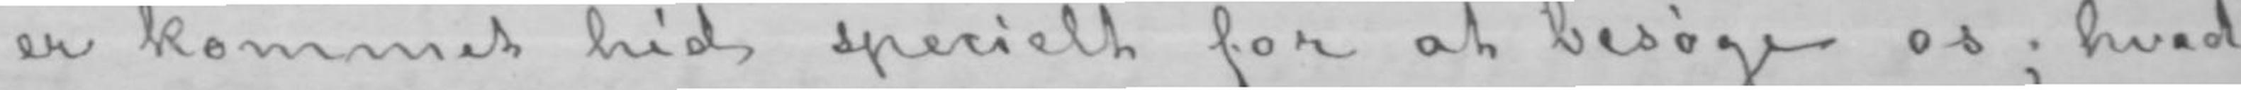er kommet hid specielt for at besøge os; hvad
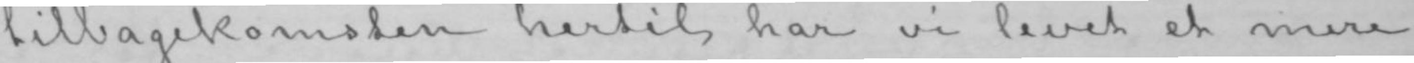tilbagekomsten hertil har vi levet et mere
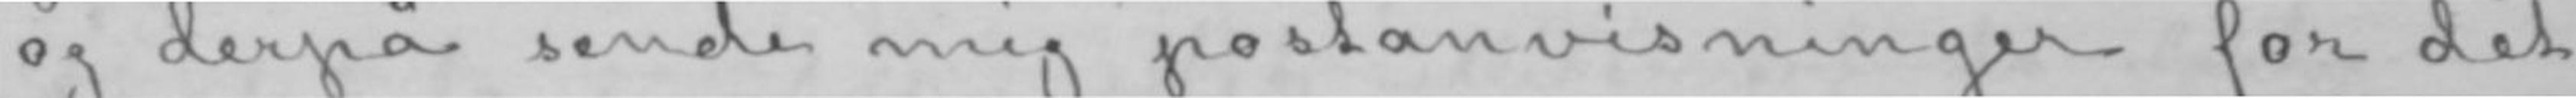og derpå sende mig postanvisninger for det
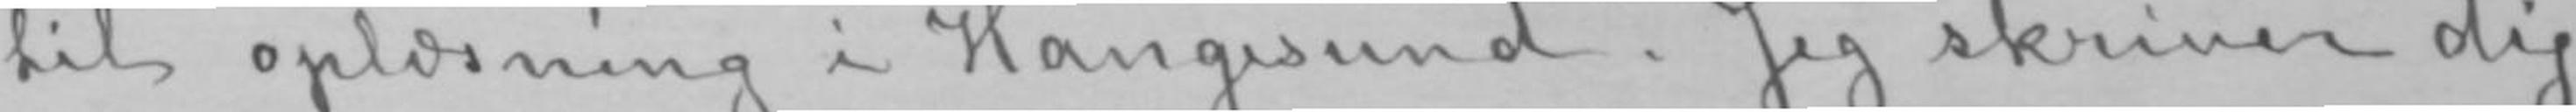til oplæsning i Haugesund. Jeg skriver dig-
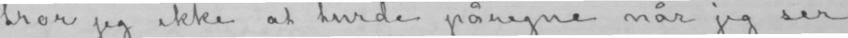tror jeg ikke at turde påregne når jeg ser
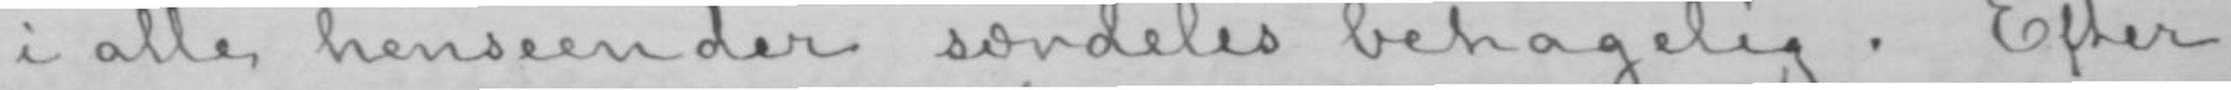i alle henseender særdeles behagelig. Efter
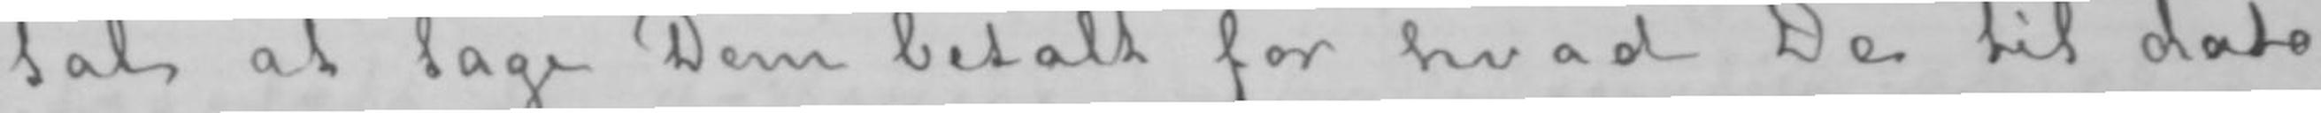tal at tage Dem betalt for hvad De til dato
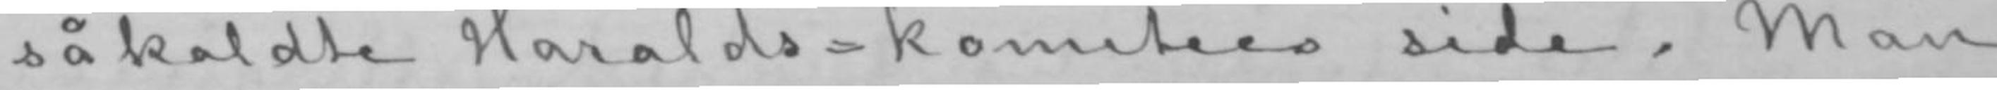såkaldte Haralds-komitees side. Man
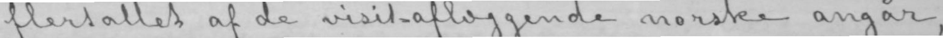flertallet af de visit-aflæggende norske angår,
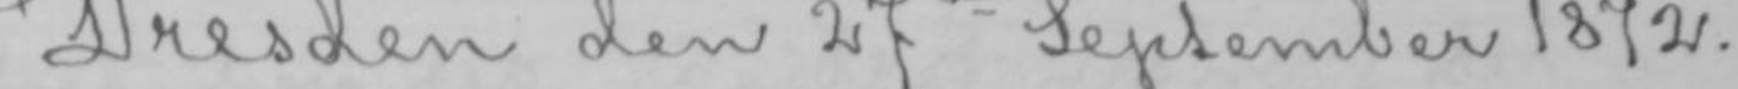Dresden den 27de September 1872.
